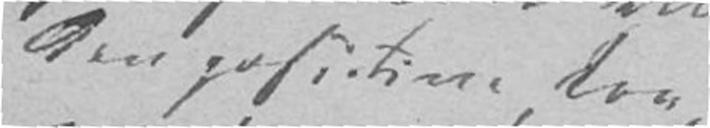den positive Lov
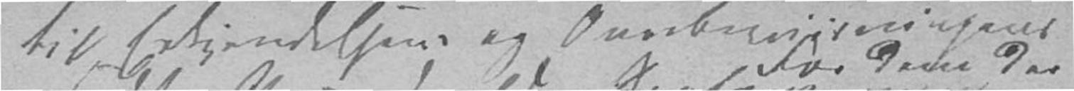til Erkjendelsens og Overbeviisningens
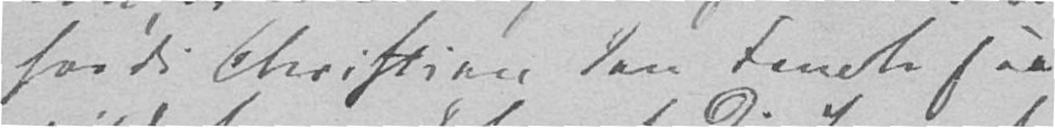fordi Christian den Femte saa
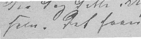selv. Det faaer
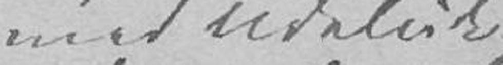med Udeluk-
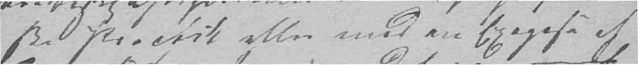ske Practik eller med en Exegese af
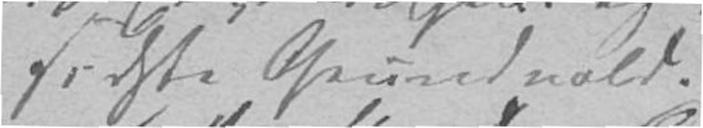sidste Grundvold.
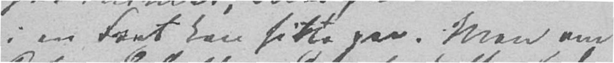i en Fart kan hitte paa. Men om
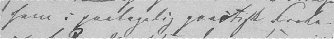ham i paatagelig practisk Forle-
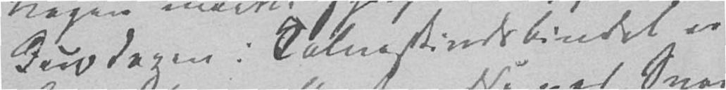Fundazen i Calveskindsbindet er
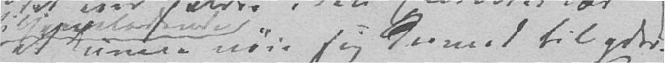at kunne nøie sig dermed til yder-
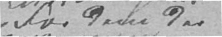For dem der
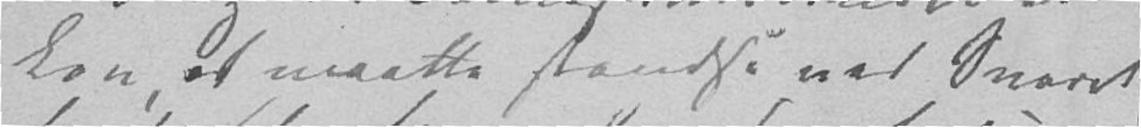Lov, at maatte standse ved Svaret
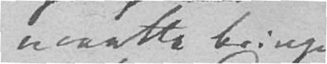maatte bringe
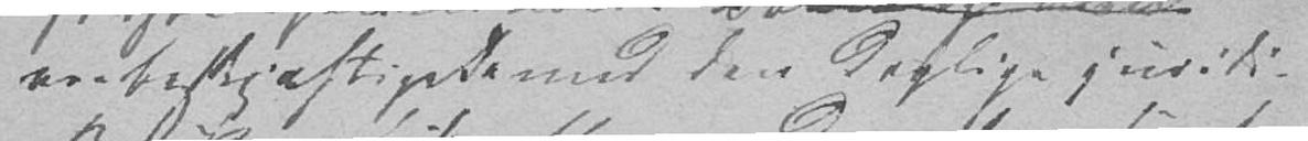ere beskjæftigede med den daglige juridi-
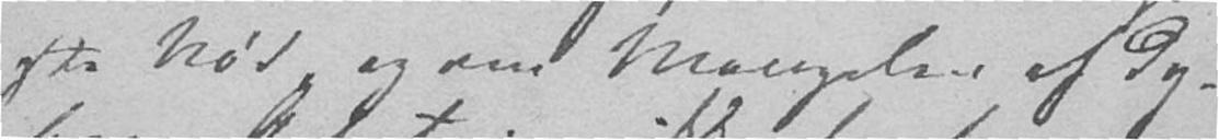ste Nød og om Mangelen af dy-
